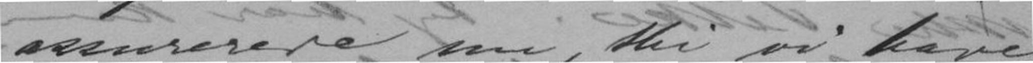assurerede nu, thi vi have
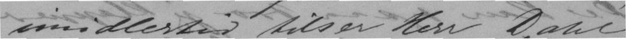imidlertid hilser Herr Dahl
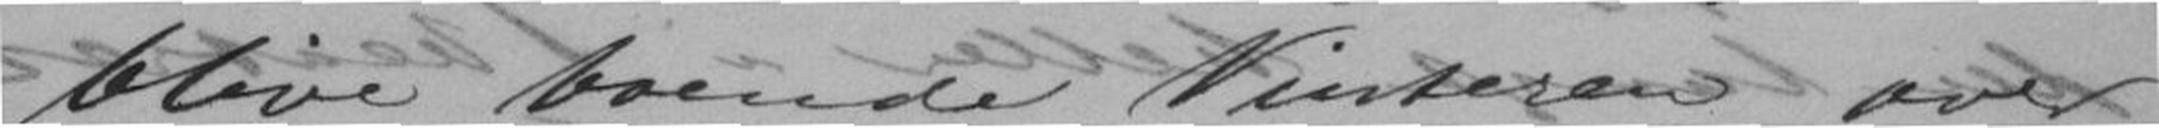blive boende Vinteren over,
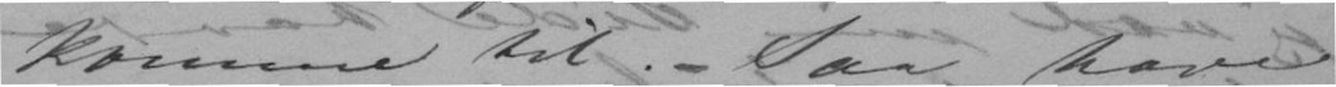komme til. - Saa have
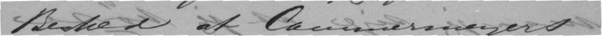Besked at Cammermeyers
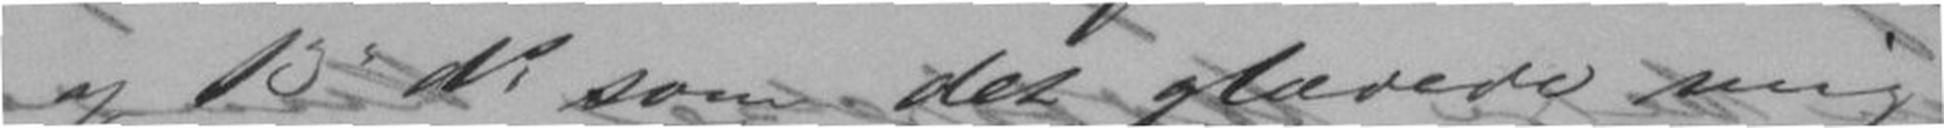af 13"ds som det glædede mig
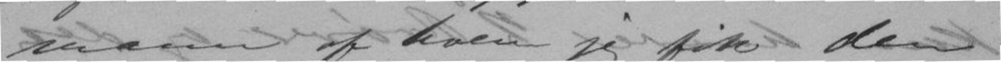mann af hvem jeg fik den
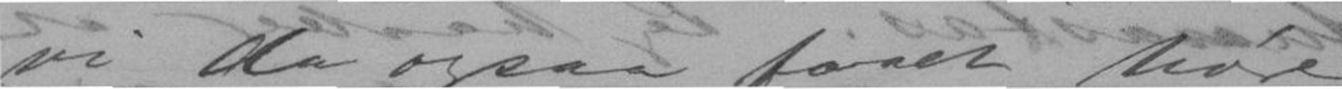vi da ogsaa faaet høre
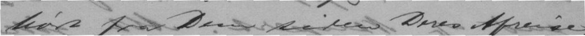hørt fra Dem siden Deres Afrejse.
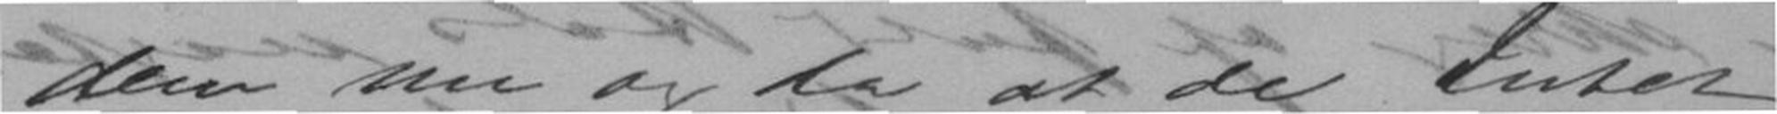den nu og da at de Intet
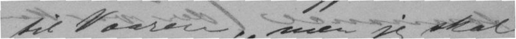til Vaaren, men jeg skal
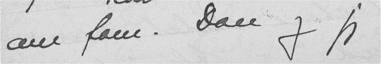om form. Don og jeg
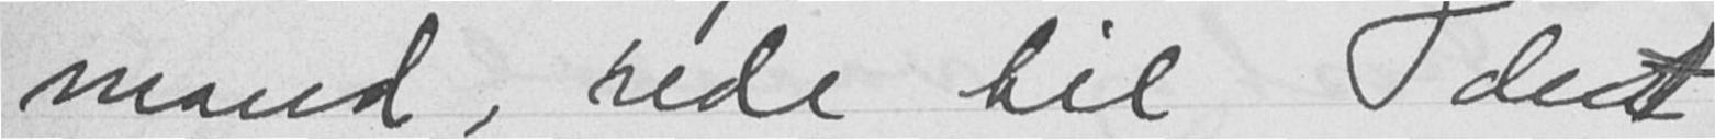mand, bede til det
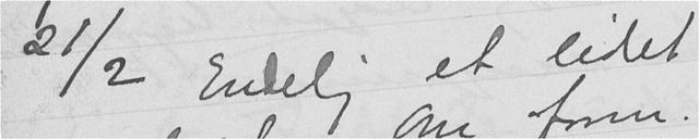21/2 Endelig et lidet
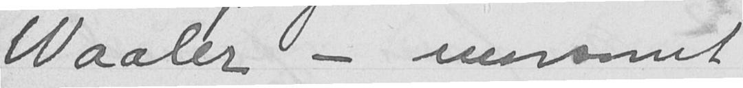Waaler - morsomt
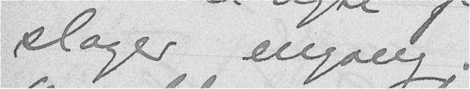slager engang.
